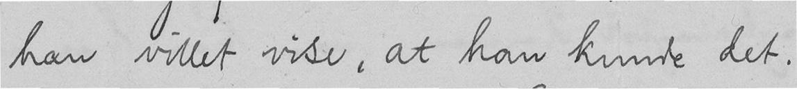han villet vise, at han kunde det.
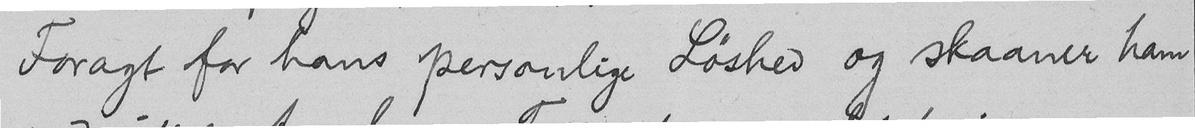Foragt for hans personlige Løshed og skaaner ham
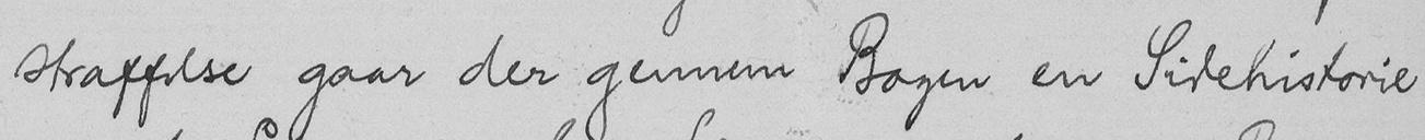straffelse gaar der gennem Bogen en Sidehistorie
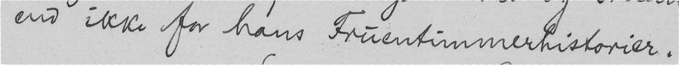end ikke for hans Fruentimmerhistorier.
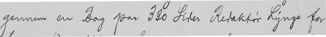gennem en Bog paa 330 Sider Redaktør Lynge for
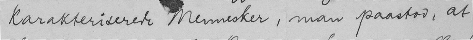karakteriserede Mennesker, man paastod, at
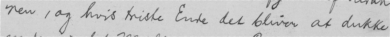ren, og hvis triste Ende det bliver at dukke
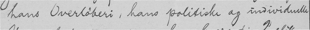hans Overløberi, hans politiske og individuelle
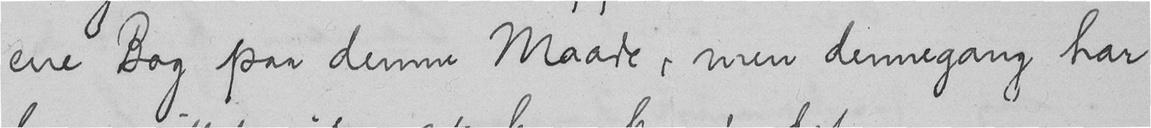ene Bog paa denne Maade, men dennegang har
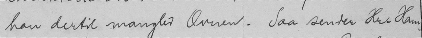han dertil mangled Ævnen. Saa sender Hr. Ham-
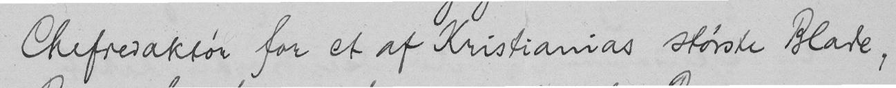Chefredaktør for et af Kristianias største Blade,
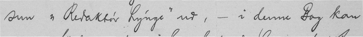sun "Redaktør Lynge" ud, - i denne Bog kan
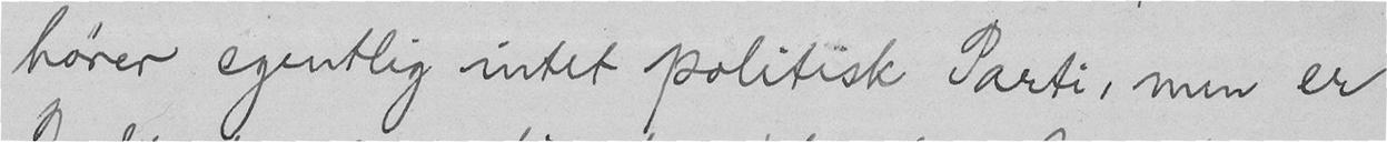hører egentlig intet politisk Parti, men er
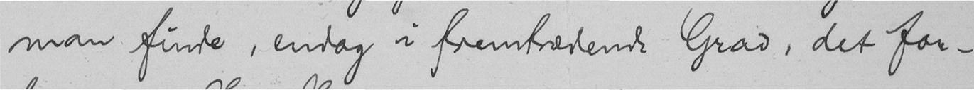man finde, endog i fremtrædende Grad, det for-
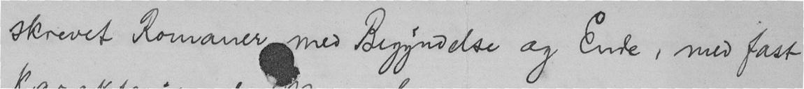skrevet Romaner med Begyndelse og Ende, med fast
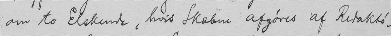om to Elskende, hvis Skæbne afgøres af Redaktø-
